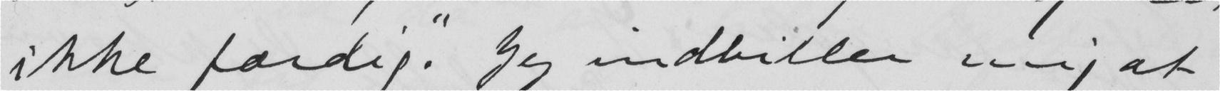ikke færdig". Jeg indbiller mig at
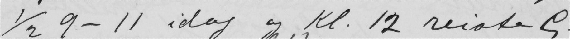1/2 9 - 11 idag og Kl. 12 reiste G.
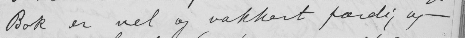Bok er vel og vakkert færdig og -
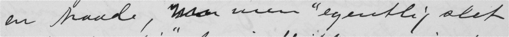en Maade,  men "egentlig slet
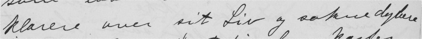klarere over sit Liv og sokne dybere
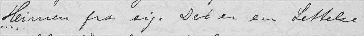Heimen fra sig. Det er en Lettelse
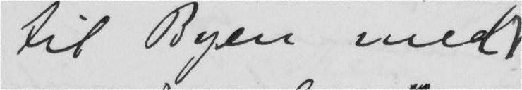til Byen med
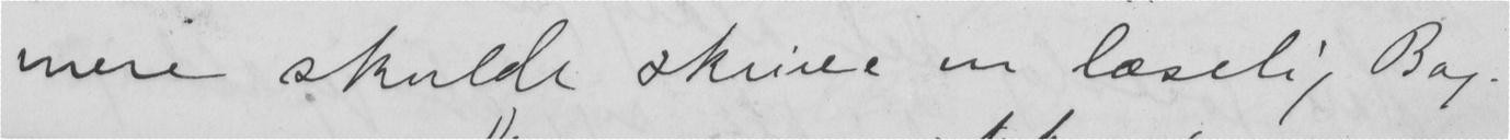mere skulde skrive en læselig Bog.
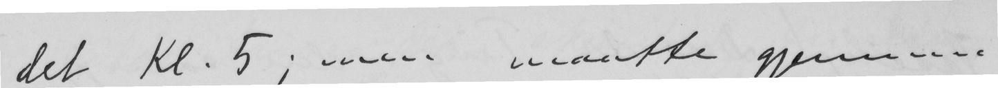det Kl. 5; men maatte gjemmme
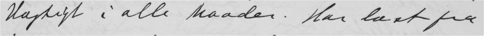Hæftigt i alle Maader. Har læst fra
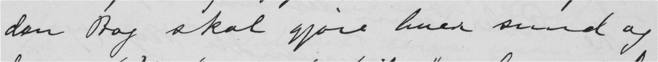den Bog skal gjøre hver sund og
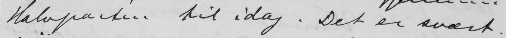Halvparten til idag. Det er svært.
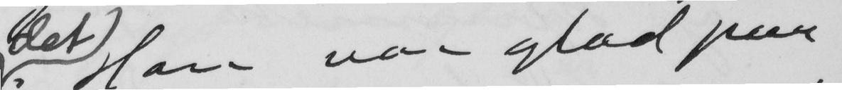. Han var glad paa
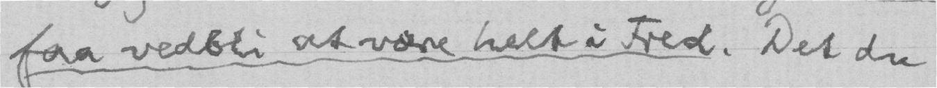faa vedbli at være helt i Fred. Det du
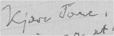Kjære Tore,
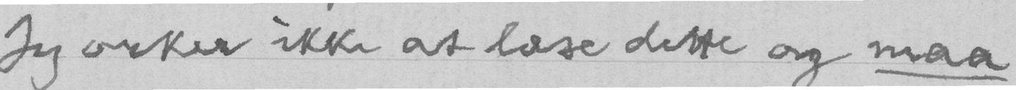Jeg orker ikke at læse dette og maa
